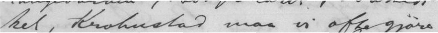ket, Krohnstad maa vi ofte gjøre,
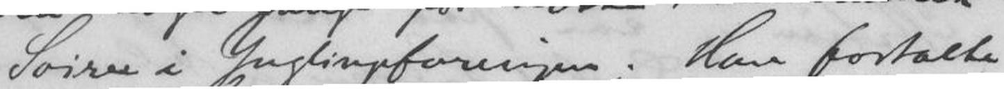Soiree i Ynglingeforeningen. Han fortalte
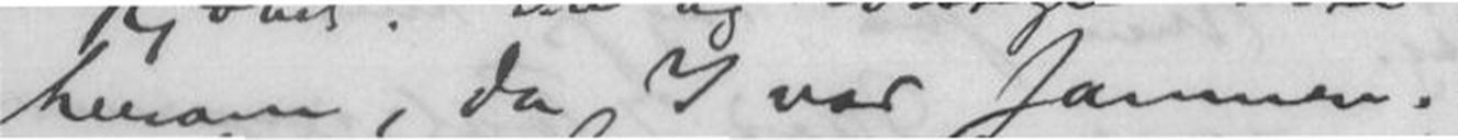herom, da I var sammen.
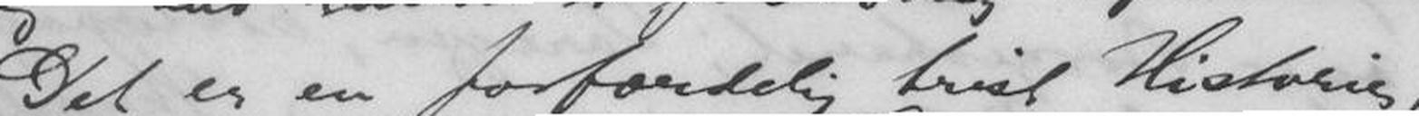Det er en forfærdelig trist Historie,
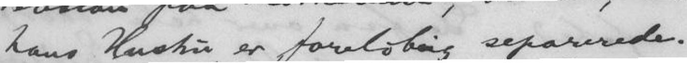og hans Hustru er foreløbig separerede.
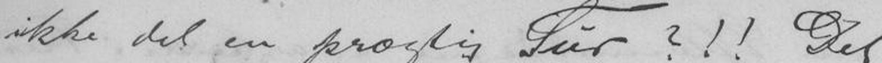ikke det en prægtig Tur?!! Det
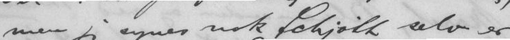men jeg synes nok Schjøtt selv er
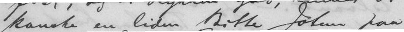kanske en liden Bitte Jotun paa
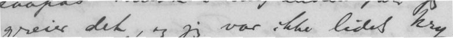greier det, og jeg var ikke lidet kry
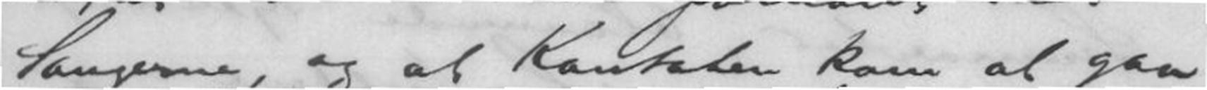Sangerne, og at Kantaten kom at gaa
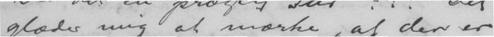glæder mig at mærke, at der er
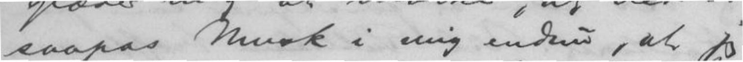saapas Musk i mig endnu, at jeg
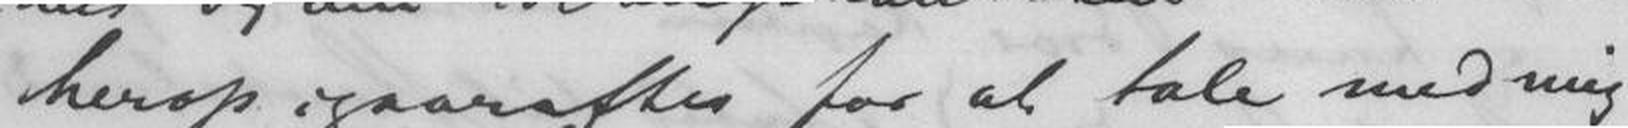herop igaaraftes for at tale med mig
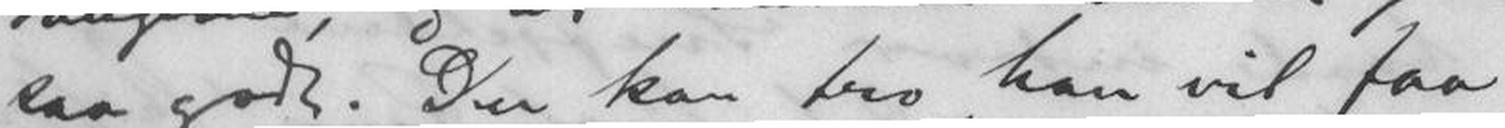saa godt. Du kan tro, han vil faa
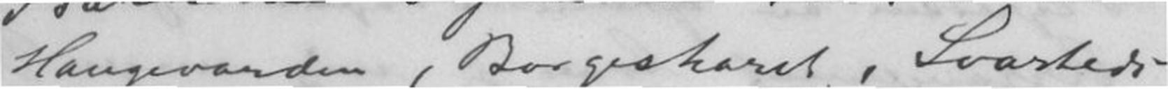Haugevarden, Borgeskaret, Svartedi-
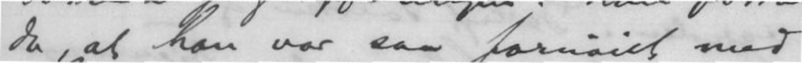da, at han var saa fornøiet med
